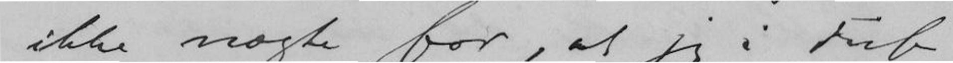ikke nægte for, at jeg i disse
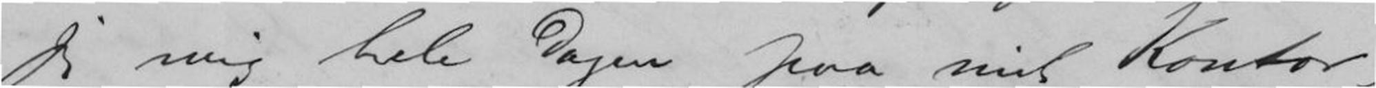jeg mig hele Dagen paa mit Kontor,
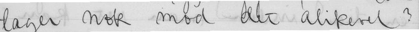tager nok mod det alikevel?
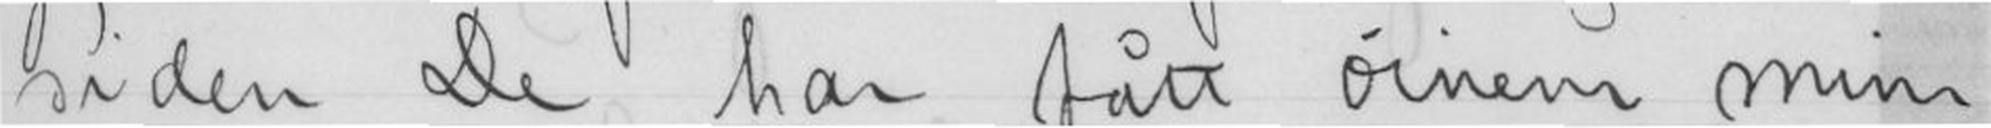siden De har fått øinene mine
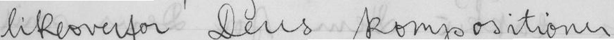likeoverfor Deres kompositioner
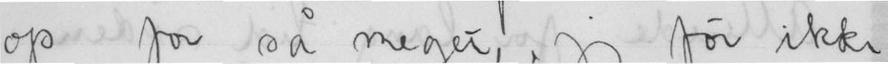op for så meget, jeg for ikke
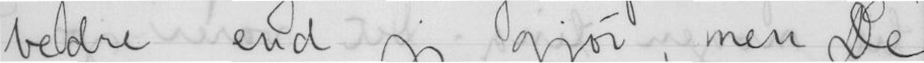bedre end jeg gjør, men De
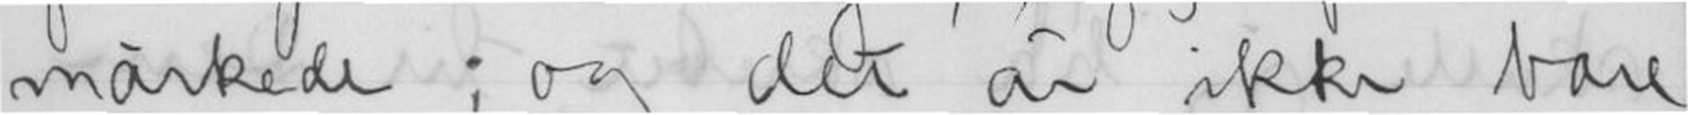màrkede; og det er ikke bare
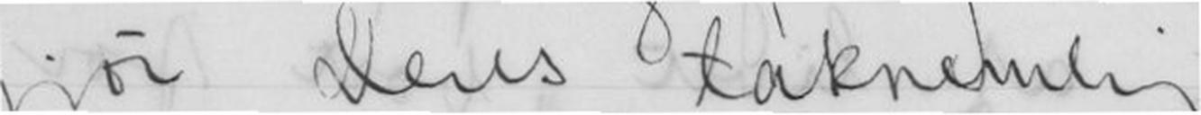gjør Deres taknemlige -
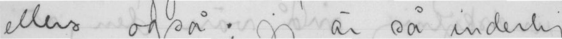ellers også; jeg er så inderlig
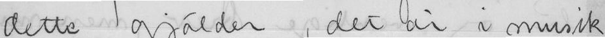dette gjälder, det er i musik
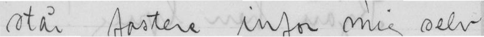jeg står fastere infor mig selv
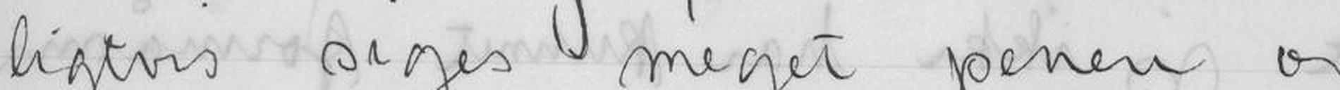ligvis siges meget penere og
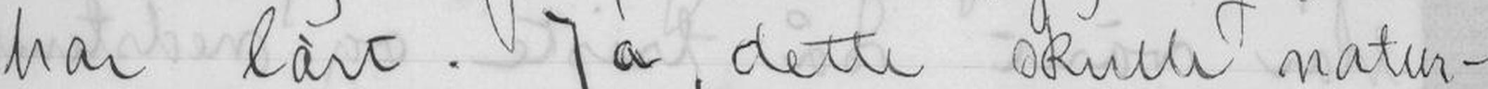har lärt. Ja, dette skulle natur-
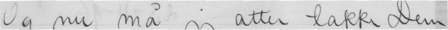Og nu må jeg atter takke Dem
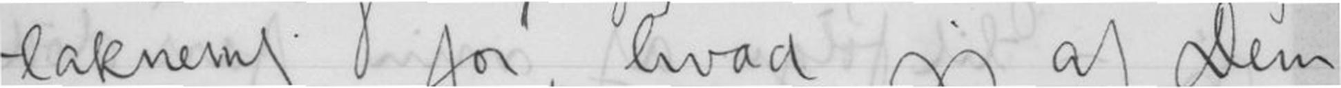taknemlig for, hvad jeg af Dem
